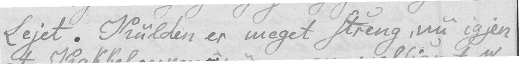Lejet. Kulden er meget streng, nu igjen
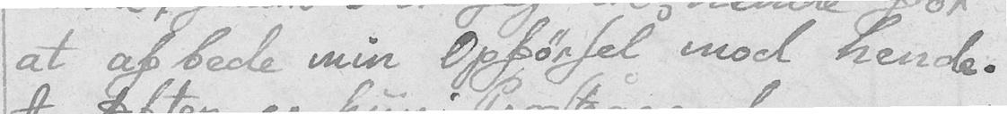at afbede min Opførsel mod hende.
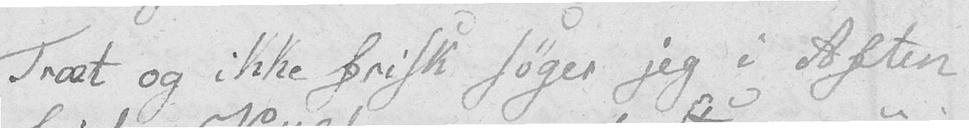Træt og ikke frisk søger jeg i Aften
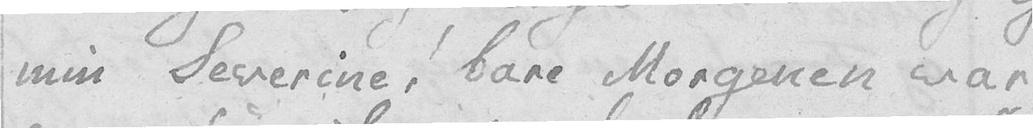min Severine! bare Morgenen var
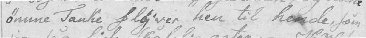ømme Tanke flyver hen til hende, som
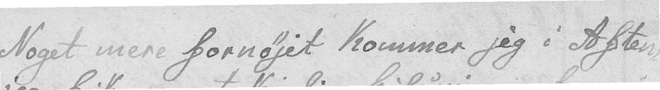Noget mere fornøjet kommer jeg i Aften,
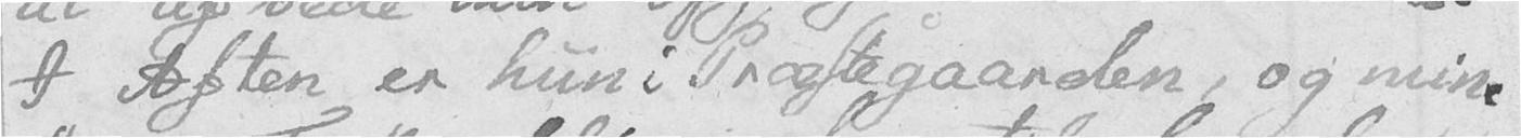I Aften er hun i Præstegaarden, og mine
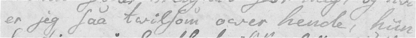er jeg saa tvilsom over hende, hun
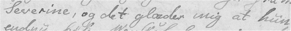Severine, og det glæder mig at hun
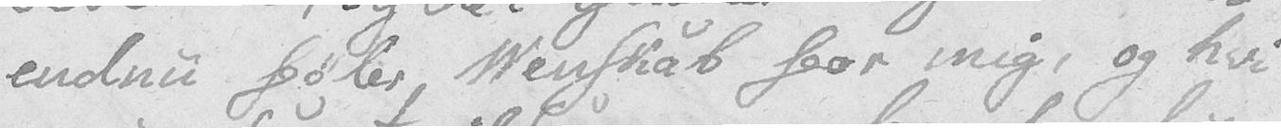endnu føler Venskab for mig, og hvi
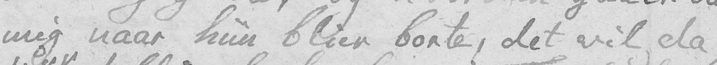mig naar hun blier borte, det vil da
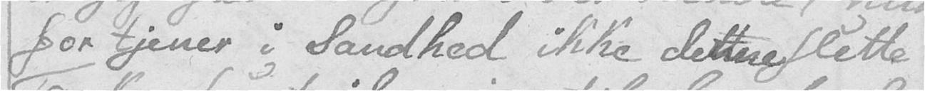fortjener i Sandhed ikke denne slette
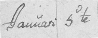Januari 5te
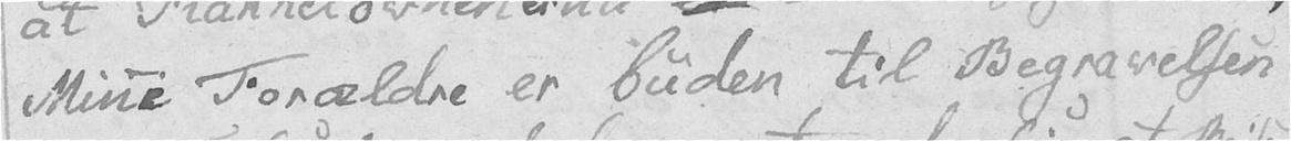Mine Forældre er buden til Begravelsen
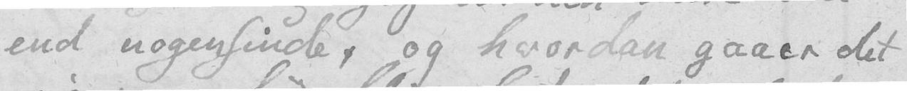end nogensinde, og hvordan gaaer det
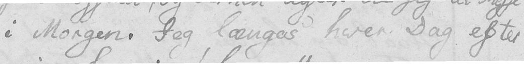i Morgen. Jeg længes hver Dag efter
